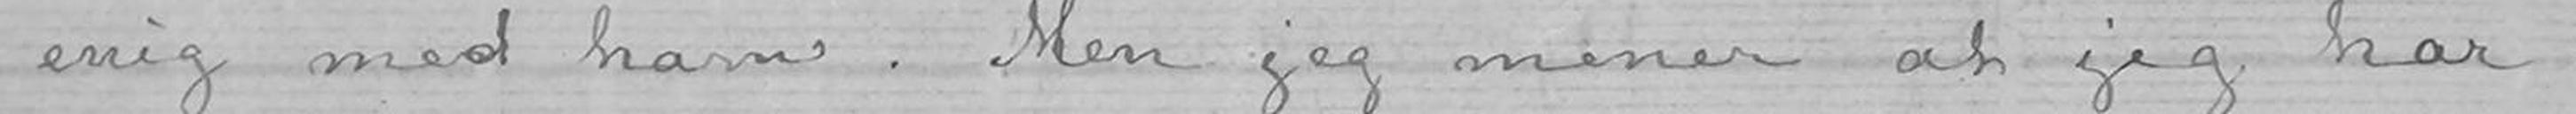enig med ham. Men jeg mener at jeg har
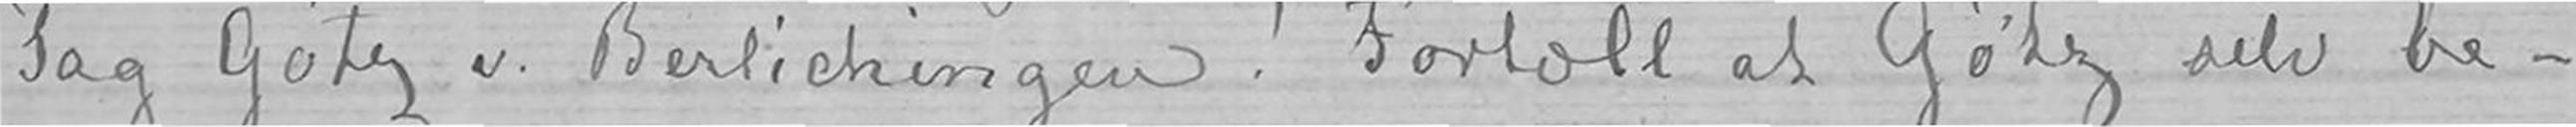Tag Götz v. Berlichingen! Fortæll at Götz selv be-
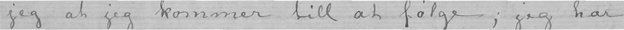jeg at jeg kommer till at følge; jeg har
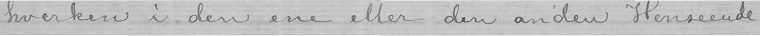hverken i den ene eller den anden Henseende.
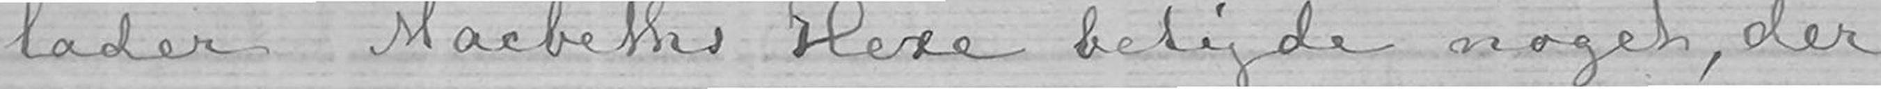lader Macbeths Hexe betyde noget, der
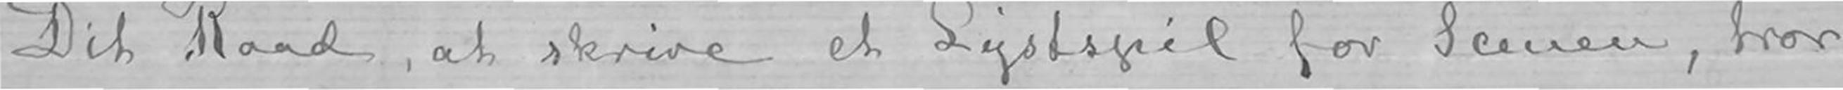Dit Raad, at skrive et Lystspil for Scenen, tror
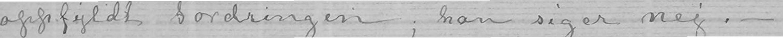oppfyldt Fordringen; han siger nej.-
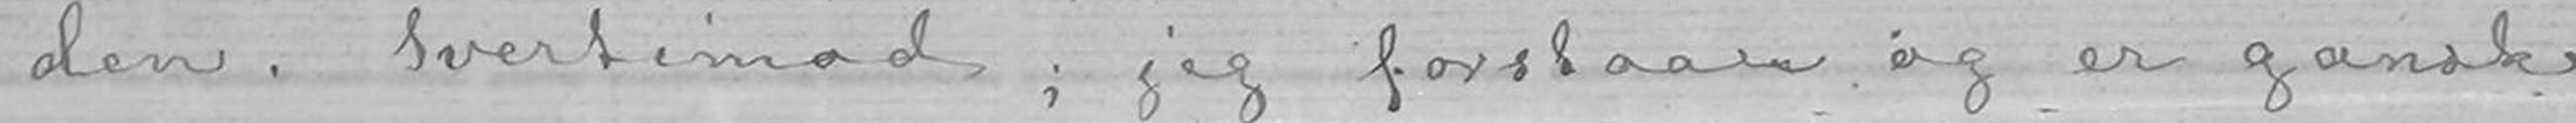den. Tvertimod; jeg forstaar og er ganske
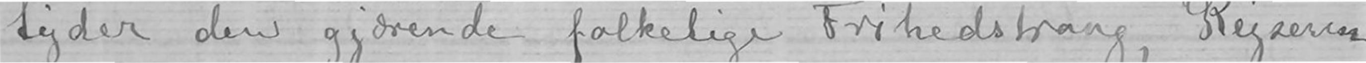tyder den gjærende folkelige Frihedstrang, Kejseren
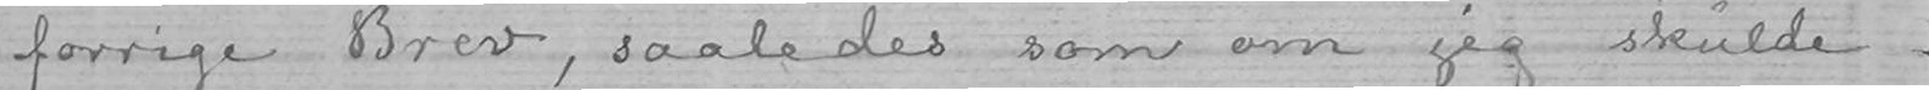forrige Brev, saaledes som om jeg skulde
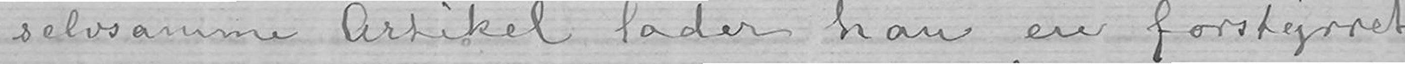selvsamme Artikel lader han en forstyrret
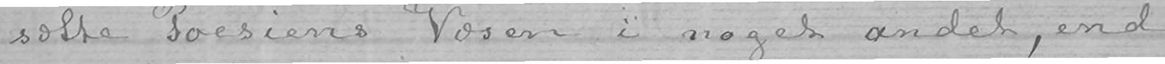sætte Poesiens Væsen i noget andet, end
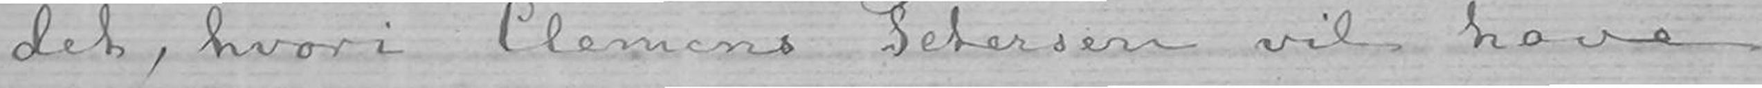det, hvori Clemens Petersen vil have
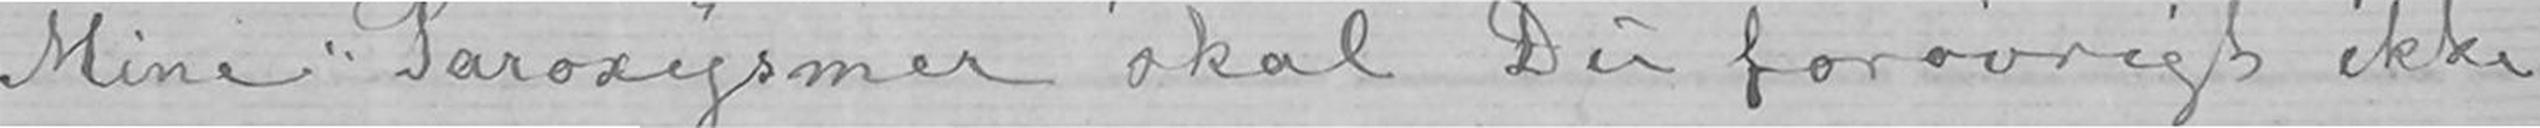Mine «Paroxysmer» skal Du forøvrigt ikke
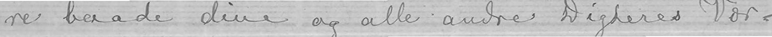re baade dine og alle andre Digteres Vær-
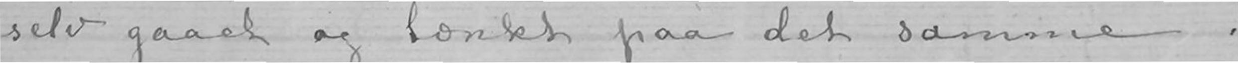selv gaaet og tænkt paa det samme.
